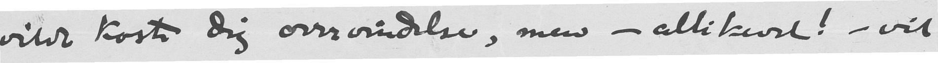vilde koste dig overvindelse, men - allikevel! - vil
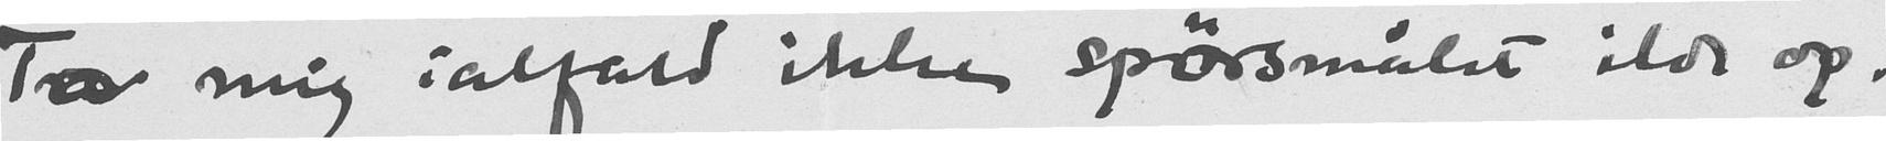Ta mig ialfald ikke spørsmålet ilde op.
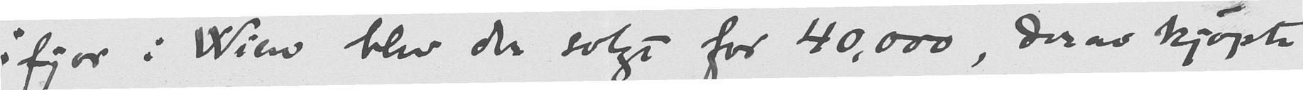i fjor i Wien blev der solgt for 40,000, derav kjøpte
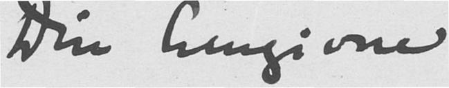Din hengivne
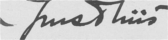Jens Thiis
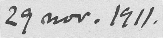29 nov. 1911.
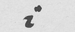i
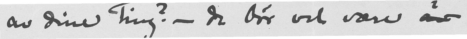av dine ting? - de bør vel være av
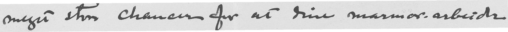meget store chancer for at dine marmor-arbeider
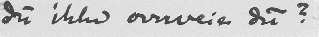du ikke overveie det?
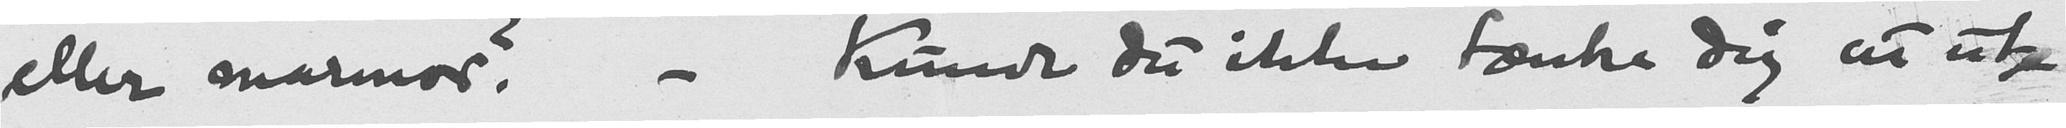eller marmor? - Kunde du ikke tænke dig at ut-
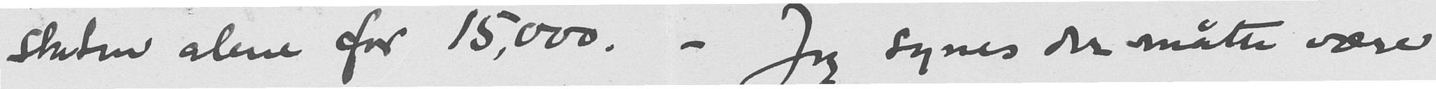staten alene for 15,000. - Jeg synes der måtte være
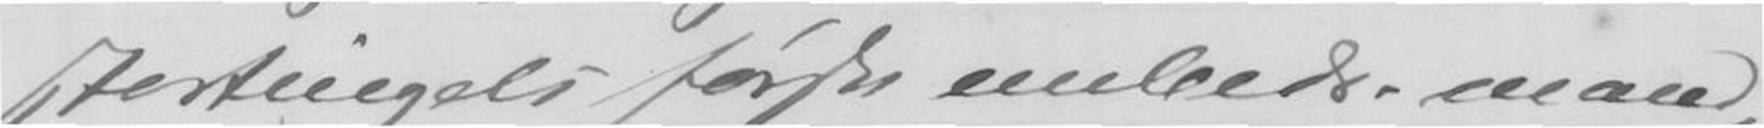stortingets første embedsmand,
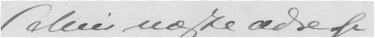(Min næste Adresse
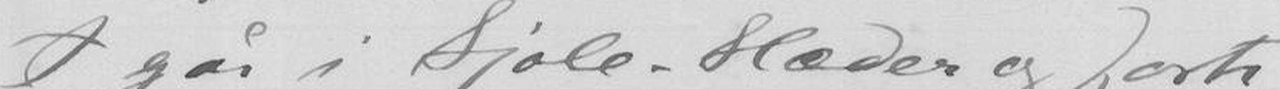I går i kjole-klæder og sorte
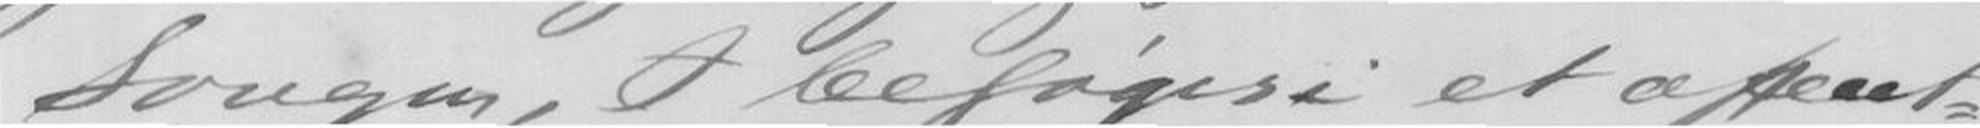kongen, I besøger i et offent-
